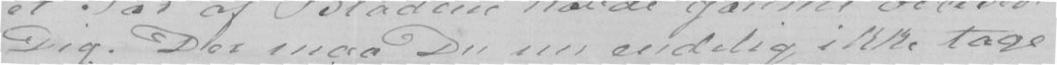Dig. Der maa Du nu endelig ikke tage
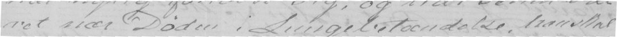ret nær Døden i Lungebetændelse, han skal
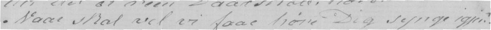Naar skal vel vi faae høre Dig spørge igjen?
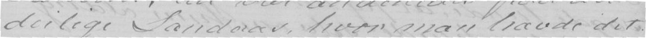deilige Landaas, hvor man havde det
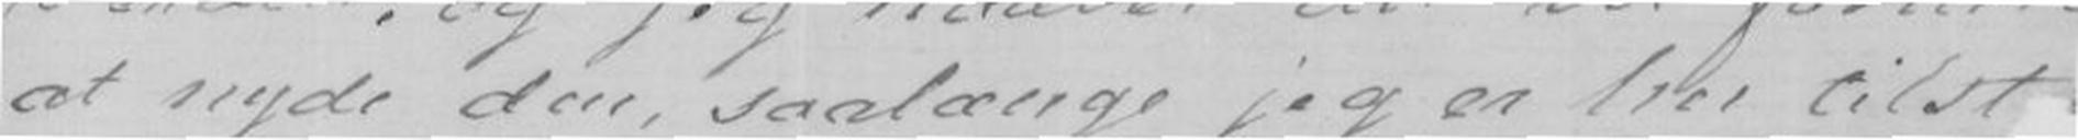at nyde den, saalænge jeg er her tilst
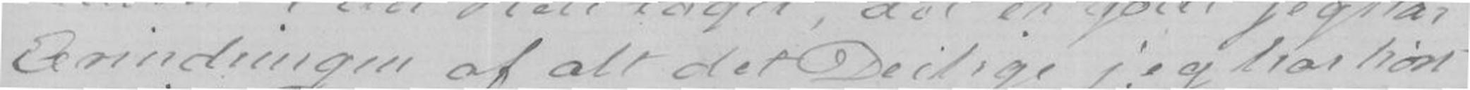Erindringen af alt det Deilige jeg har hørt
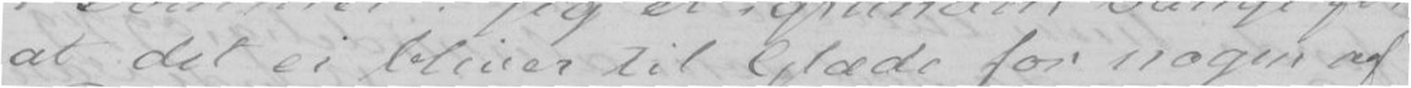at det ei bliver til Glæde for nogen af
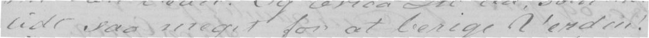lidt saa meget for at berige Verden!
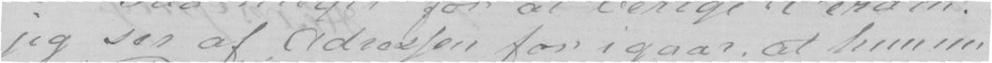jeg ser af Adressenfor igaar, at hun nu
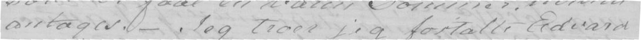antages. - Jeg troer jeg fortalte Edvard
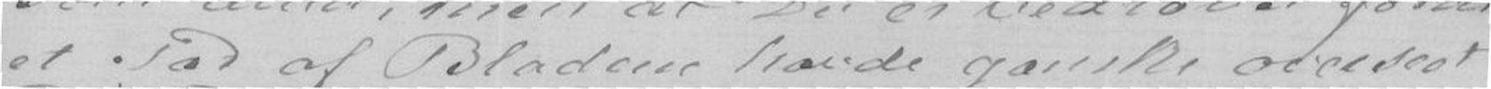et Par af Bladerne havde ganske overseet
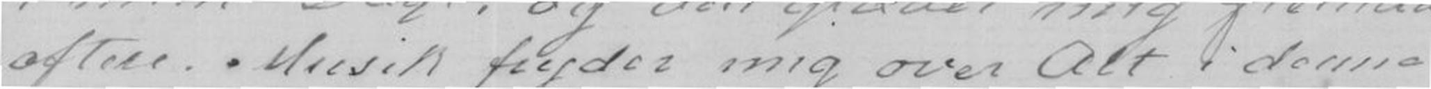oftere. Musik fryder mig over Alt i denne
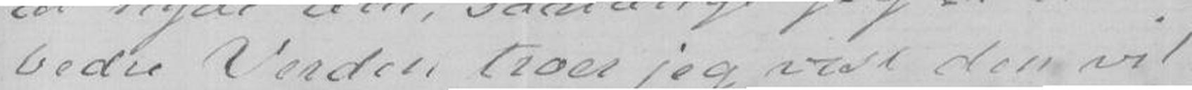bedre Verden troer jeg vise den vil bl
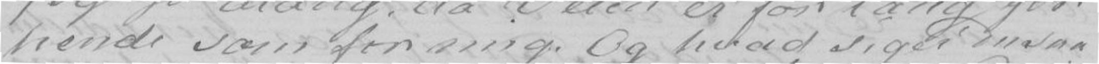hende som for mig. Og hvad siger Du saa
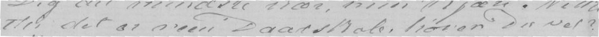thi det er reen Daarskab, hører Du vel?
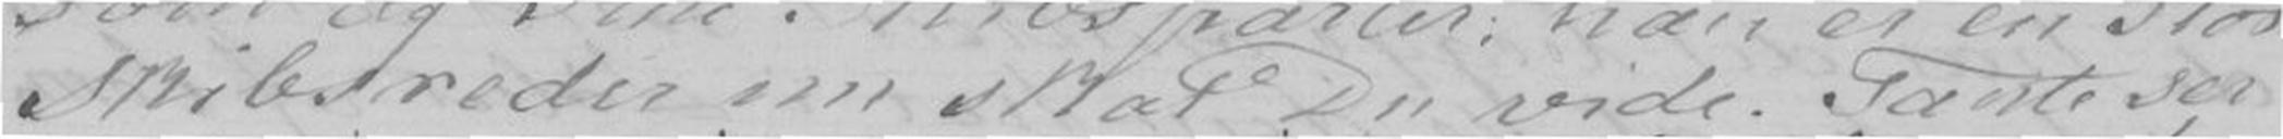Skibsreder nu skal Du vide. Tante ser
